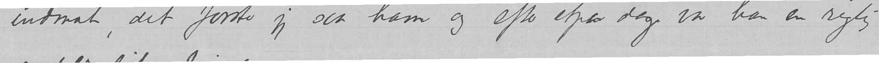indmat, det første jeg saa ham og efter etpar dager var han en rigtig
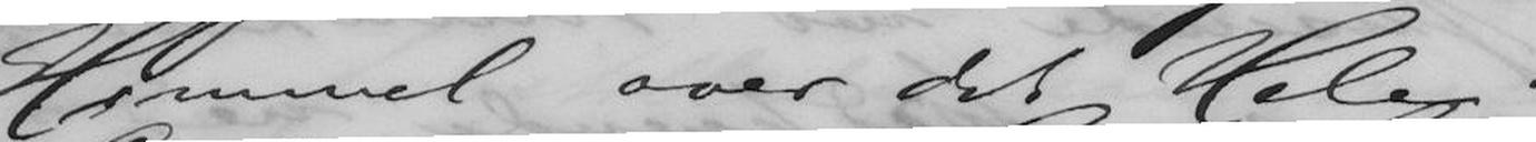Himmel over det Hele.
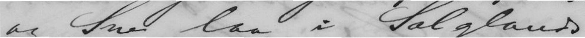og Sne laa i Solglands
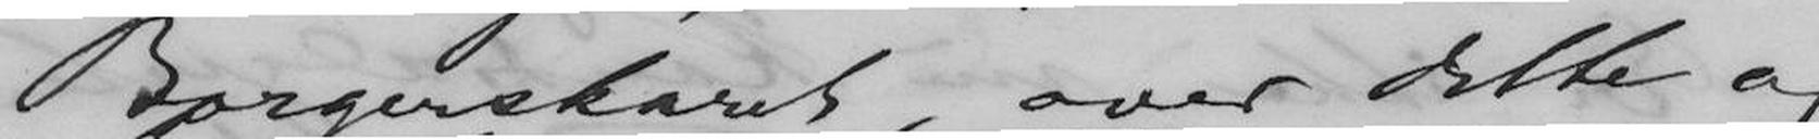Borgerskaret, over dette og
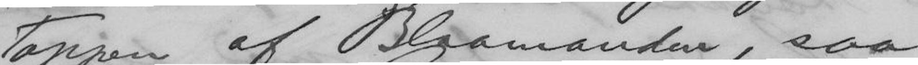Toppen af Blaamanden, saa
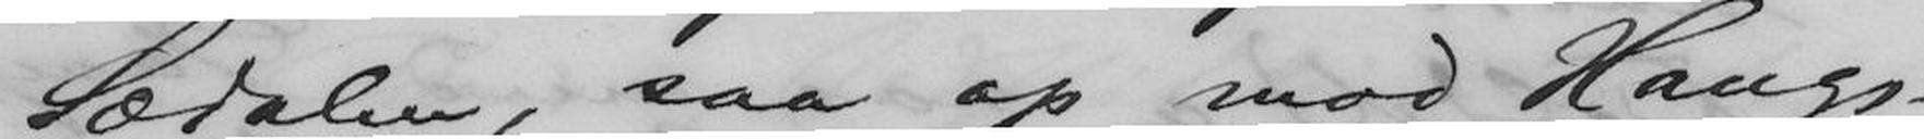Sædalen, saa op mod Haugs-
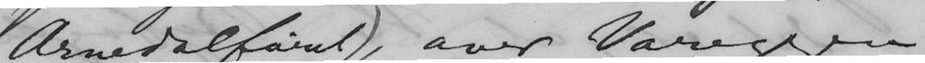Arnedalfaret), over Vareggen
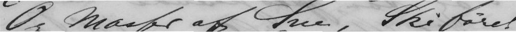Og Masser af Sne, Skiføret
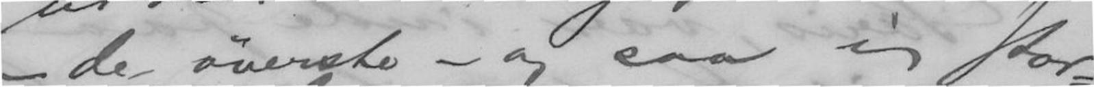- de øverste - og saa i stor-
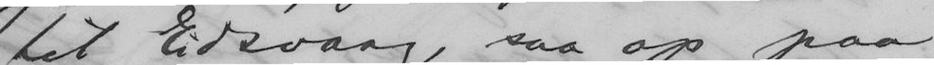til Eidsvaag, saa op paa
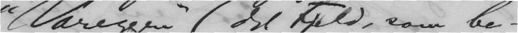"Vareggen" (det Fjeld, som be-
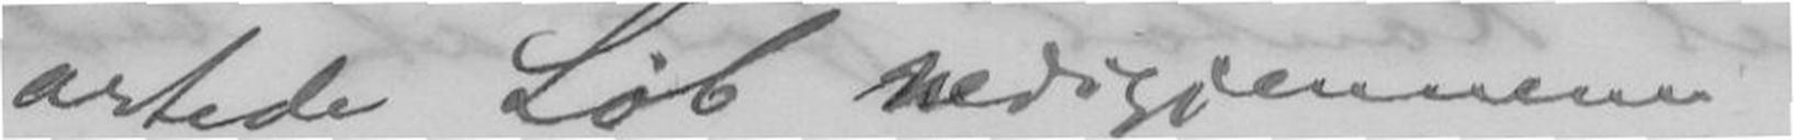artede Løb nedgjennem
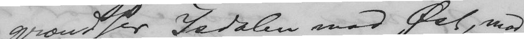grændser Isdalen mod Øst, mod
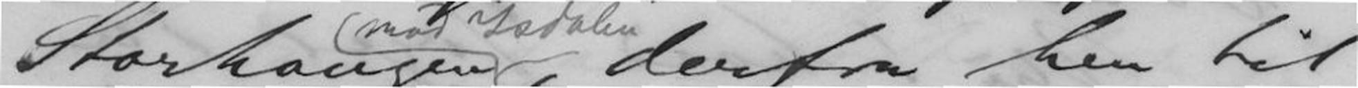Storhaugen, derfra hen til
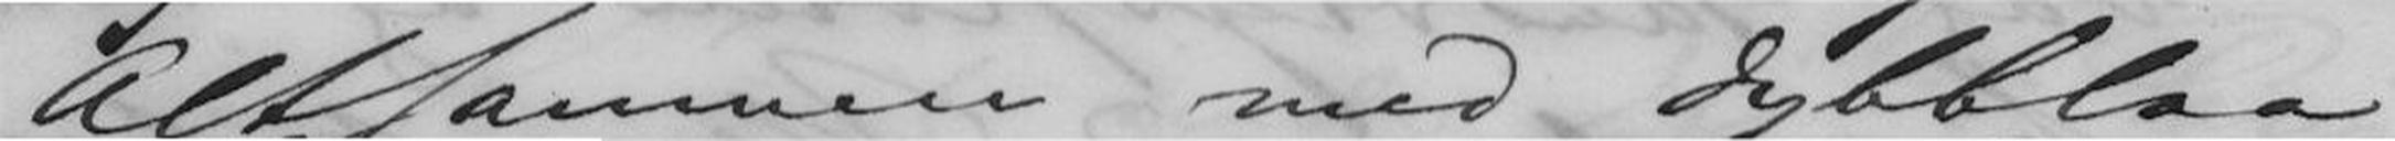Altsammen med dybblaa
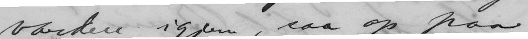varden igjen, saa op paa
